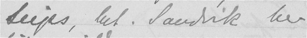Seips, lnt. Sandvik her
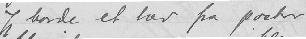Jeg havde et brev fra pastor
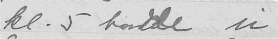kl. 5 havde vi
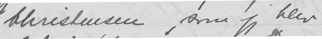Christensen, som jeg blev
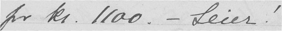for kr. 1100. - Seier!
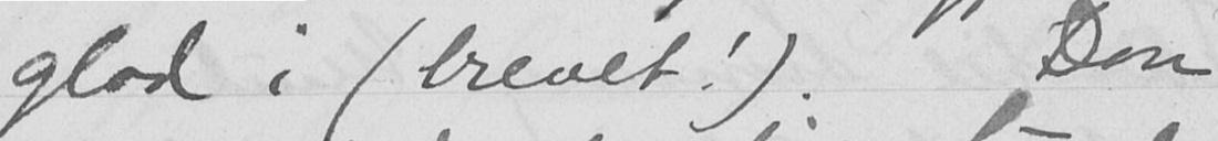glad i (brevet!) Don
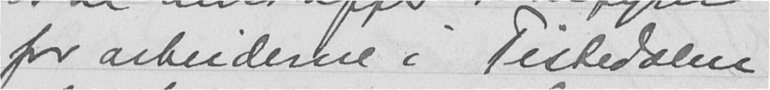for arbeiderne i Tistedalen
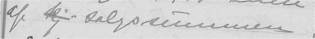af kjø salgssummen.
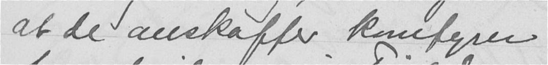at de anskaffer komfyrer
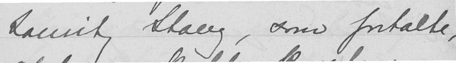Lauritz Stang, som fortalte,
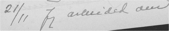21/11 Jeg arbeided om
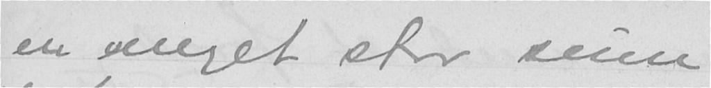en meget stor sum
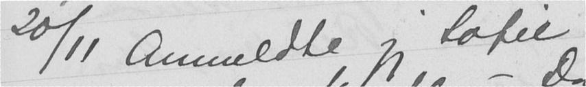20/11 Anmeldte jeg Sofie
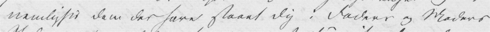nemlighed dem der have staaet Dig i Faders og Moders
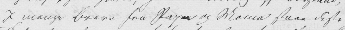I mange Breve fra Papa og Mama staae disse
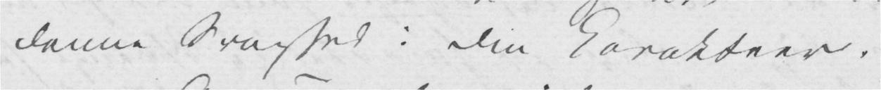denne Svaghed i din Carakteer.
